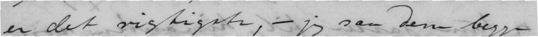er det vigtigste, - jeg saa dem begge
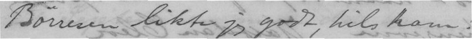Børresen likte jeg godt, hils ham!
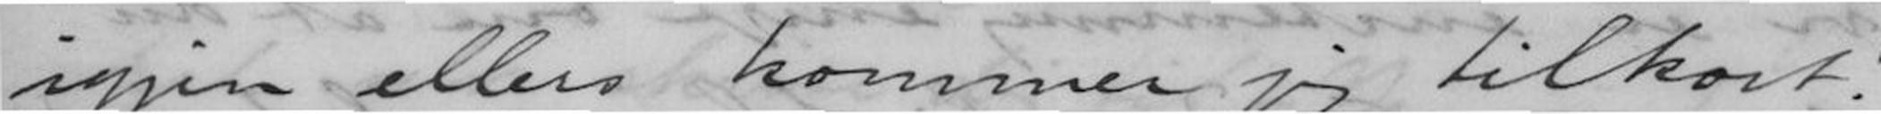igjen ellers kommer jeg tilkort!
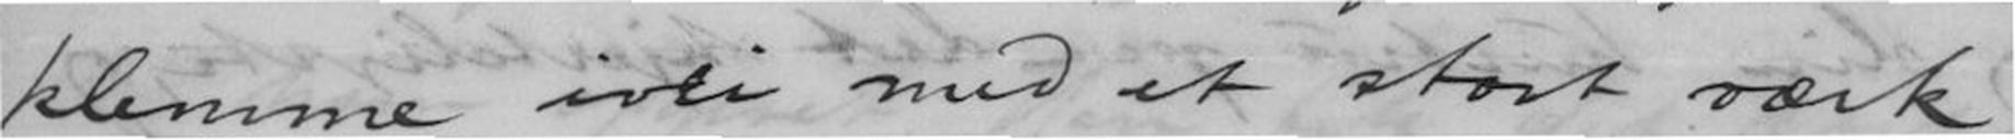klemme ivei med et stort værk
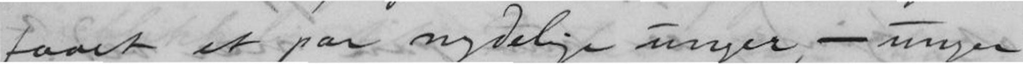faaet et par nydelige unger, - unger
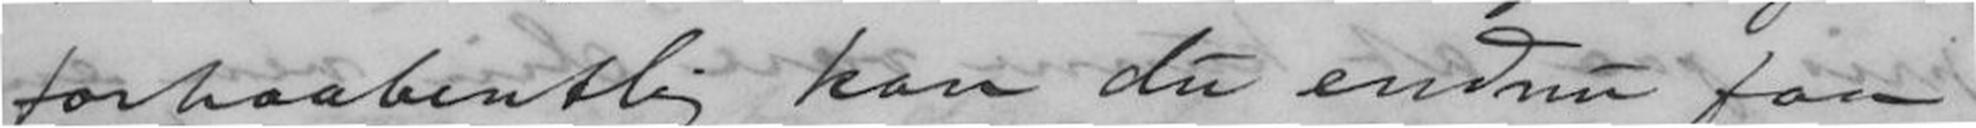forhaabentlig kan du endnu faa
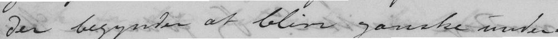der begynder at blive ganske under-
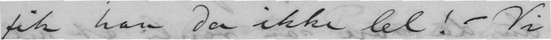fik han da ikke lel! - Vi
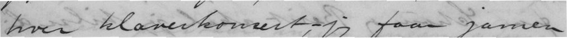hver klaverkonsert, jeg faar jamen
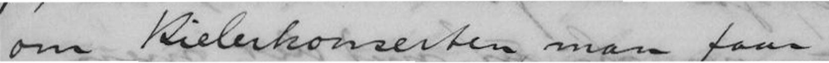om Bielerkonserten, man faar
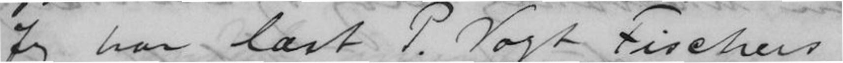Jeg har læst P. Vogt Fischers
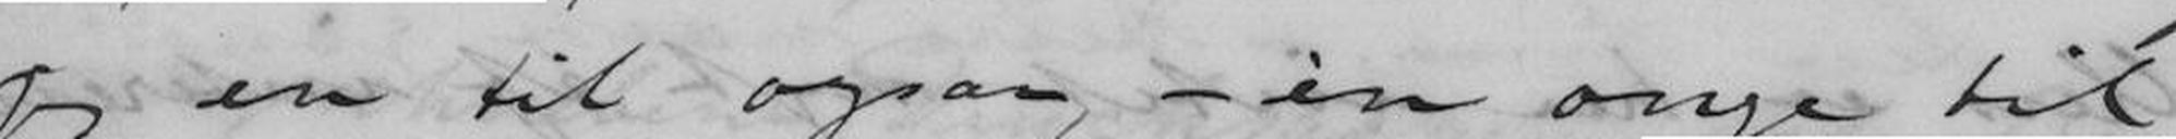jeg en til ogsaa, - en onge til
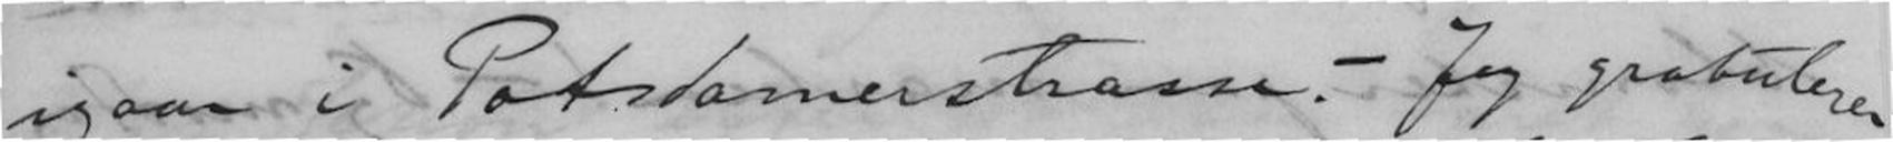igaar i Potsdamerstrasse. - Jeg gratulerer
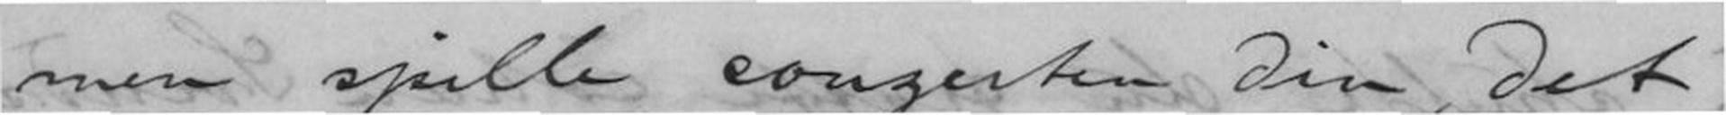men spille conzerten din, det
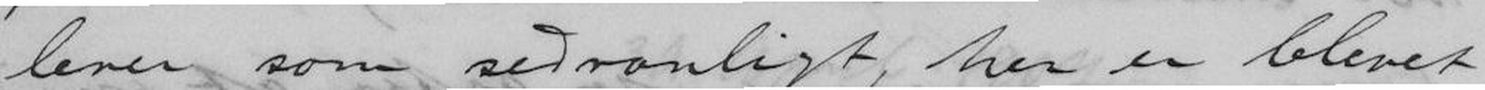lever som sedvanligt, her er blevet
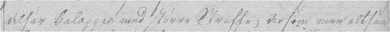delser belægges med større Straffe; dersom man altsaa
